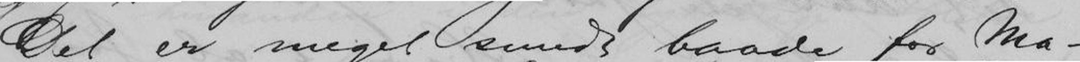Det er meget sundt baade for Ma-
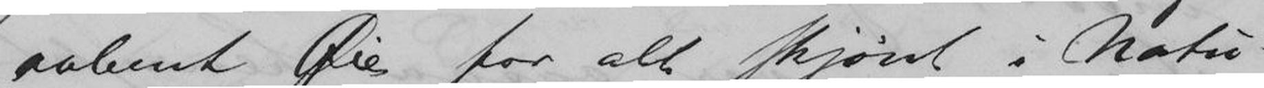aabent Øie for alt skjønt i Natu-
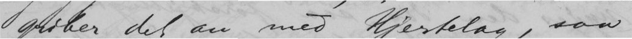griber det an med Hjertelag, saa
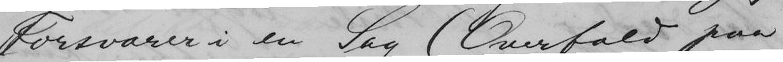Forsvarer i en Sag (Overfald paa
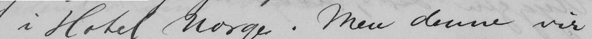i Hotel Norge. Men denne vir-
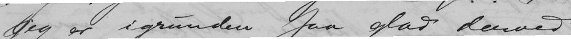jeg er igrunden saa glad derved.
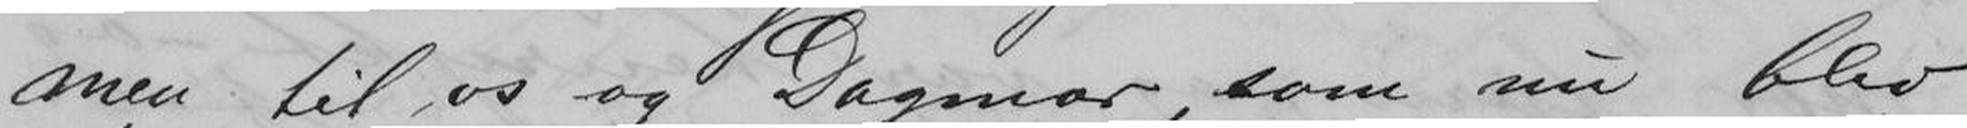men til os og Dagmar, som nu blev
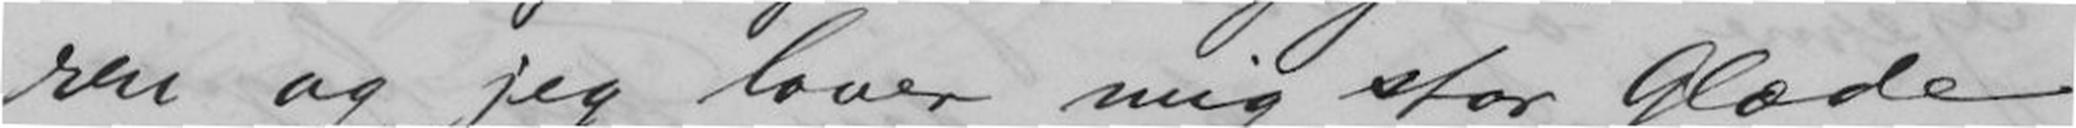ren og jeg lover mig stor Glæde
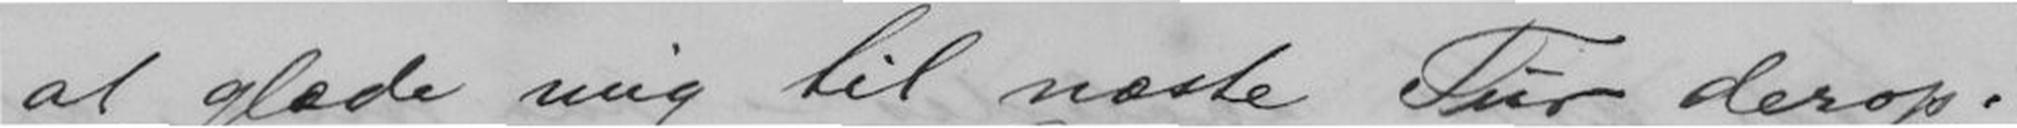at glæde mig til næste Tur derop.
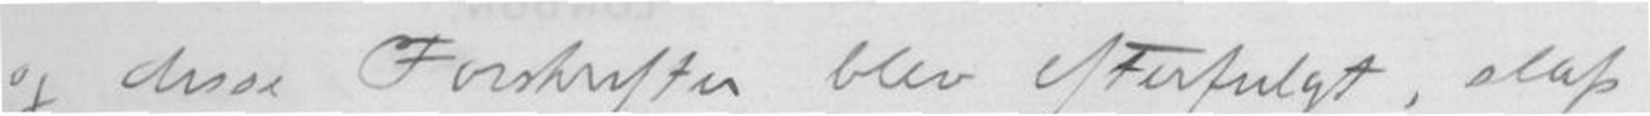og disse Forskrifter blev efterfulgt, slap
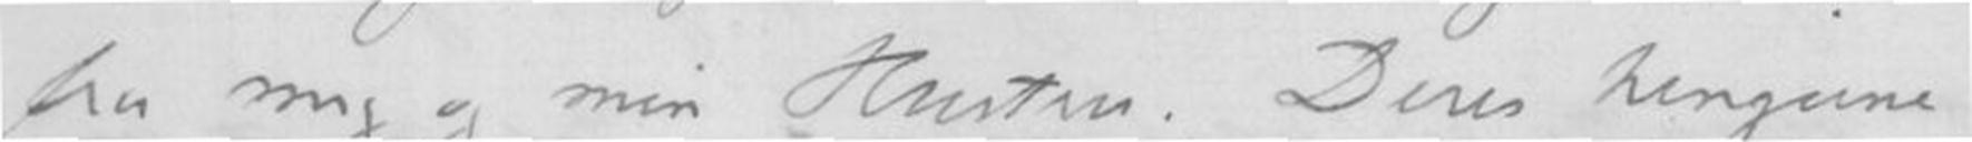fra mig og min Hustru. Deres hengivne
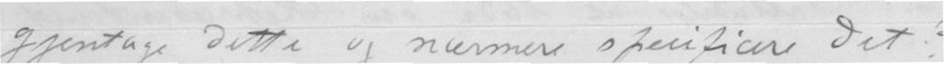gjentage dette og nærmere specifiære Det?
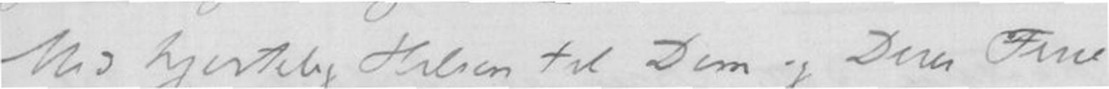Med hjertelig Hilsen til Dem og Deres Frue
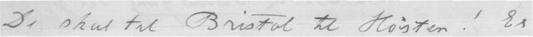De skal til Bristol til Høsten! Er
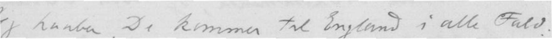Jeg haaber, De kommer til England i alle Fald.
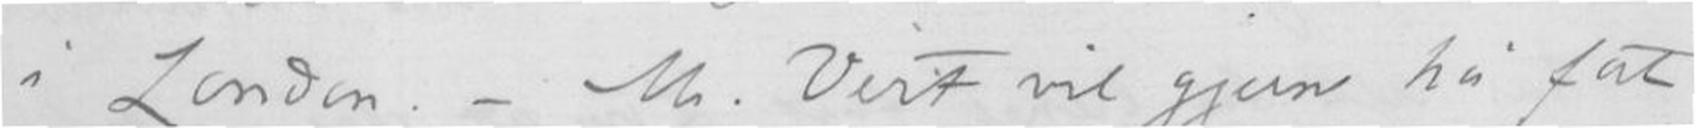i London. - Mr. Vert vil gjerne ha fat
